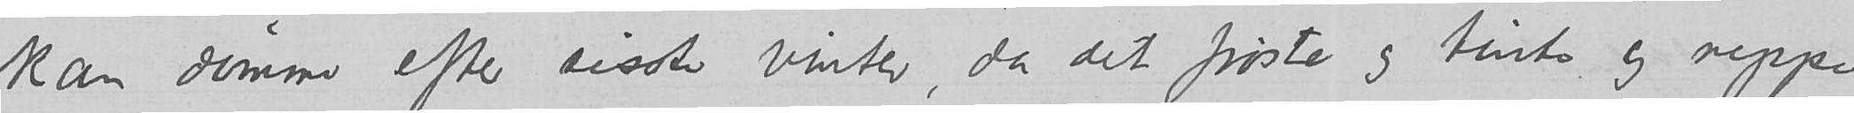kan dømme efter sisste vinter, da det frøste og tinte og neppe
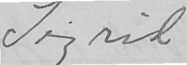Sigrid
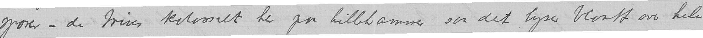sporer – de trives kolossalt her paa Lillehammer saa det lyser blaatt over hele
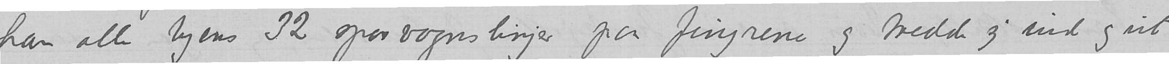han alle byens 32 sporvognslinjer paa fingrene og tredde sig ind og ut
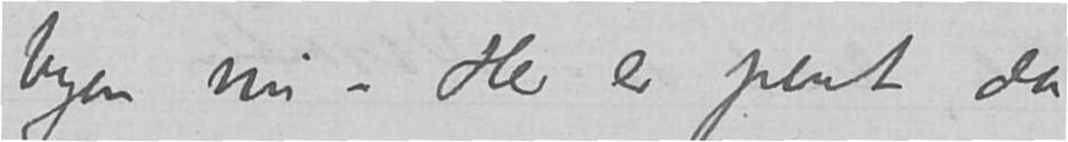byen nu – Her er pent da –.
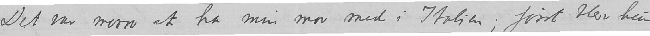Det var moro at ha min mor med i Italien; først blev hun
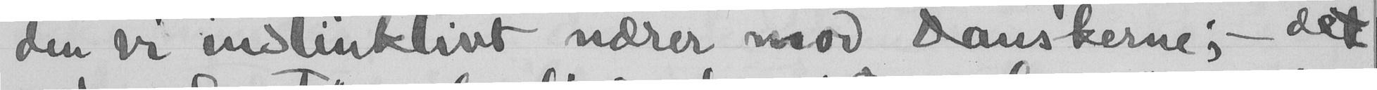den vi instinktivt nærer mod danskerne; - det
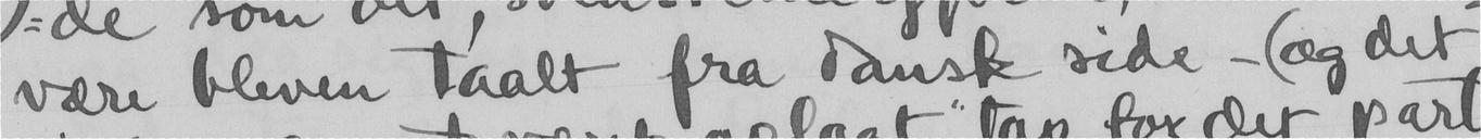være bleven taalt fra dansk side - (og det
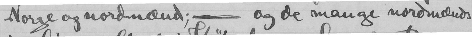Norge og nordmænd; - og de mange nordmænds
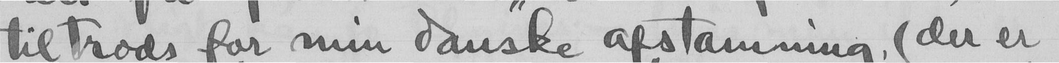tiltrods for min danske afstamning, (der er
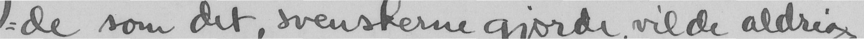-de som det, svenskerne gjorde, vilde aldrig
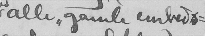alle "gamle embeds-
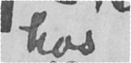hos
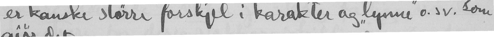er kanske større forskjel i karakter og "lynne" o.sv. som
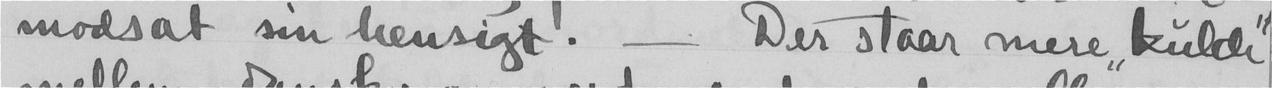modsat sin hensigt. - Der staar mere, "kulde"
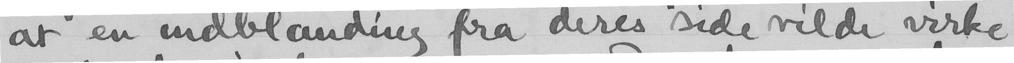at en indblanding fra deres side vilde virke
